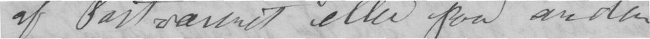af Postvæsenet eller paa anden
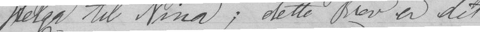Helga til Nina; dette Brev er det,
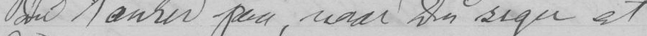Du tænker paa, naar Du siger at
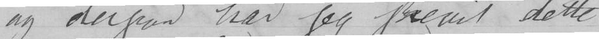og derpaa har jeg skrevet dette
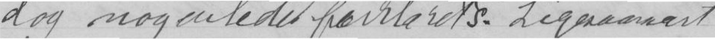dog nogenledes forklares. Ligesaasnart
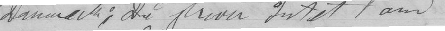Danmark; Du skriver Intet om,
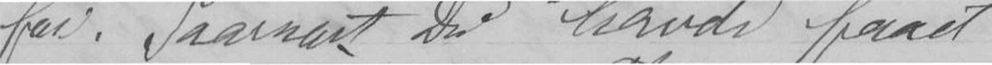før. Saasnart Du havde faaet
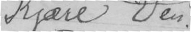Kjære Ven!
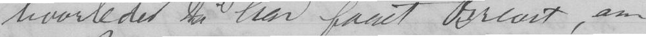hvorledes Du har faaet Brevet, om
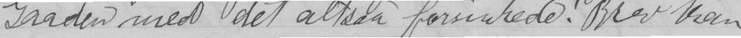Gaaden med det altsaa forsinkede! Brev kan
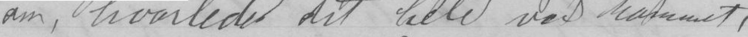om, hvorledes det hele var kommet,
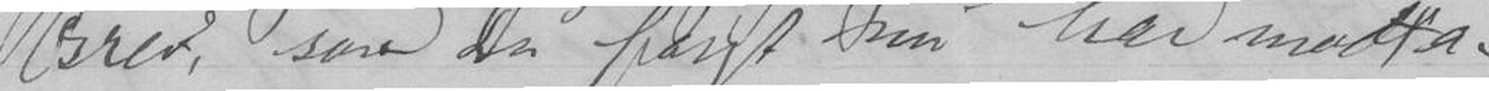Brev, som Du først nu har modta-
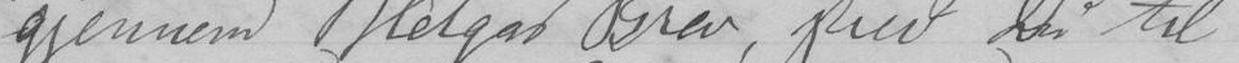gjennem Helgas Brev, skrev Du til
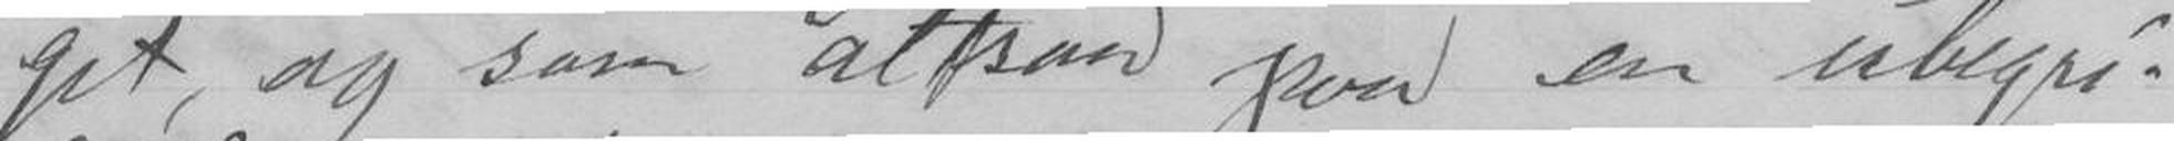get, og som altsaa paa en ubegri-
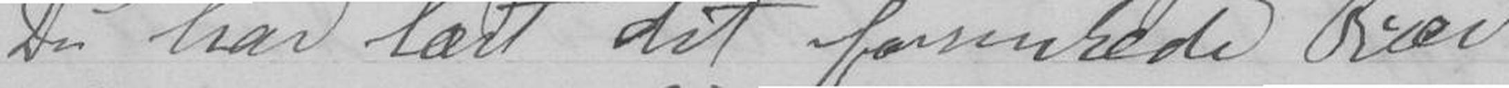Du har læst det forsinkede Brev
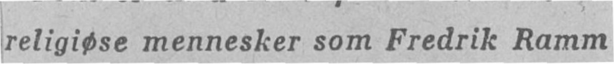religiøse mennesker som Fredrik Ramm
